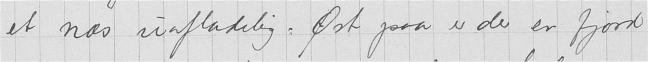et næs uafladelig. Øst paa er der en fjord
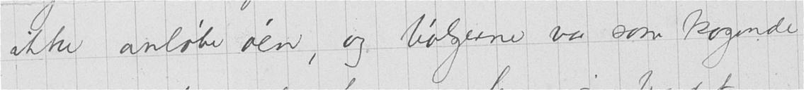ikke anløbe øen, og bølgerne var som kogende
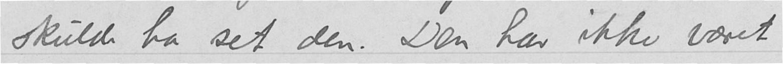skulde ha set den. Den har ikke været
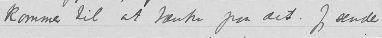kommer til at tænke paa det. Jeg sender
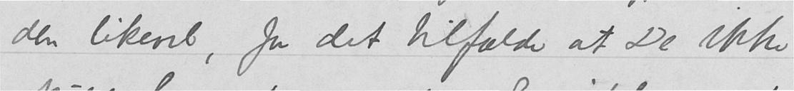den likevel, for det tilfælde at De ikke
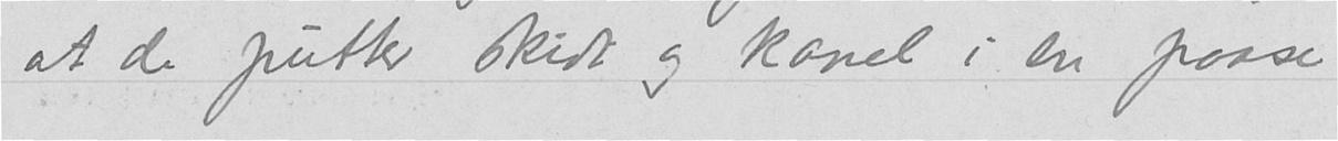at de putter skidt og kanel i en paase
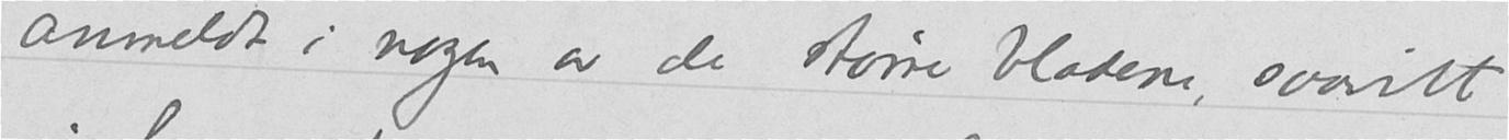anmeldt i nogen av de større bladene, saavidt
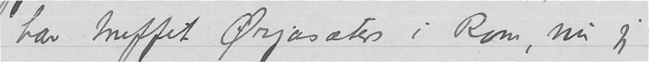har truffet Ørjasæters i Rom, nu jeg
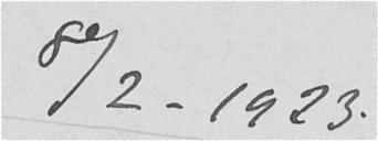8/2-1923.
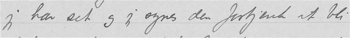jeg har set og jeg synes den fortjente at bli
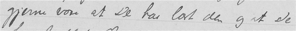gjerne være at De har læst den og at de
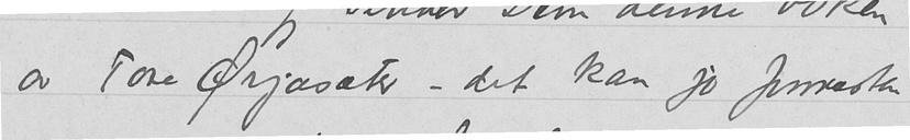av Tore Ørjasæter – det kan jo forresten
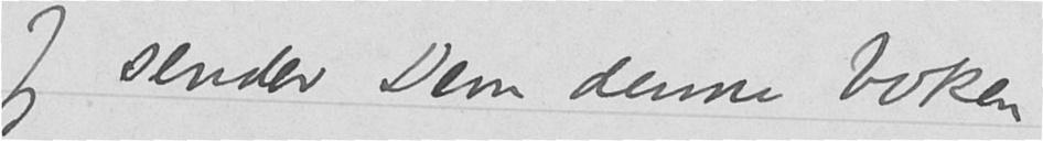Jeg sender Dem denne boken
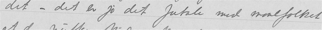det – det er jo det fatale med maalfolket
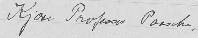Kjære Professor Paasche,
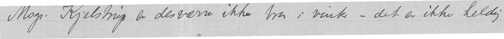Msgr. Kjelstrup er desværre ikke bra i vinter - det er ikke heldig
